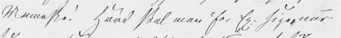Menneske! Hvad skal man for Ex. sige, når
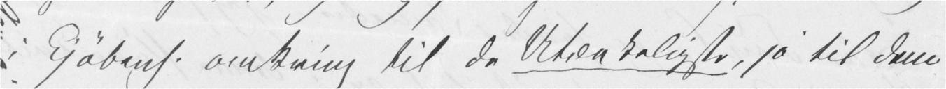i Kjøbenh. omkring til de Utænkeligste, jo til dem
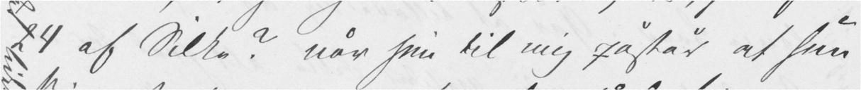24 af Silke? når hun til mig påstår at hun
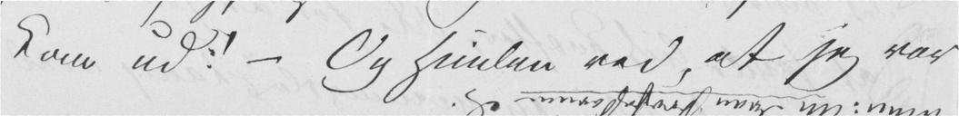kom ud! – Og Himlen ved, at jeg var
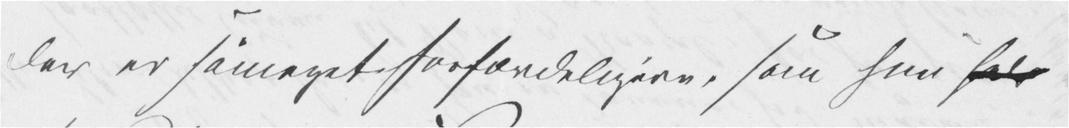der er såmeget forfærdeligere, som hun selv
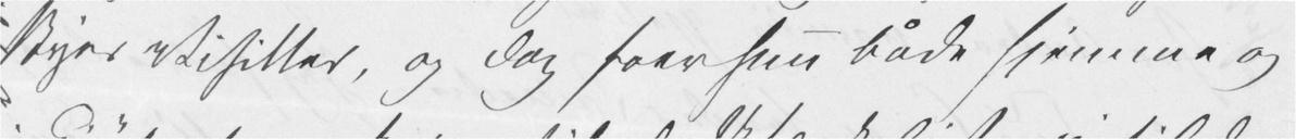skyer Visitter, og dog foer hun både hjemme og
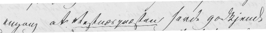engang at Vestnæspræsten havde godkjendt
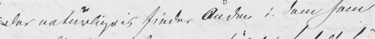der naturligvis finder Ånden i dem som
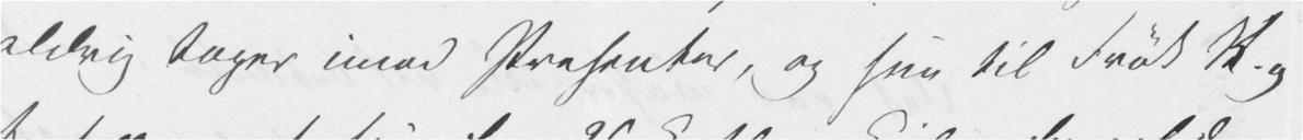aldrig tager imod Presenter, og hun til Frøk R.g
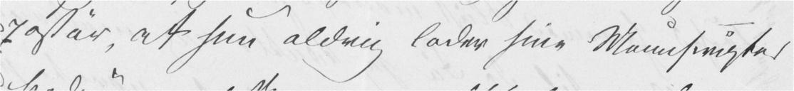pastår, at hun aldrig lader sine Manuscripter
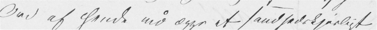Ord af hende må ægge et sandhedskjerligt
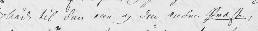både til den ene og den anden Præst,
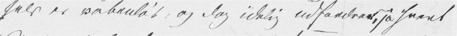selv er våbenløs, og dog idelig udfordrer, ja hvert
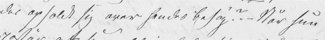der opholdt sig over hendes Besøg? – Når hun
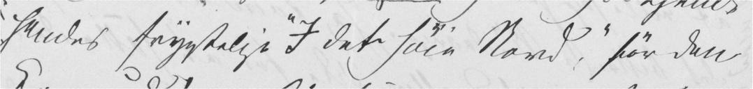hendes frygtelige "I det høie Nord," før den
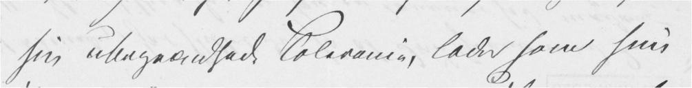sin ubegrændsede Tolerance, lader som hun
